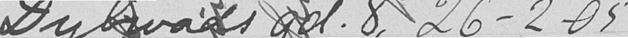Dybwads gd. 8, 26-2-05
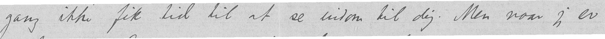gang ikke fik tid til at se indom til dig. Men naar jeg er
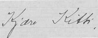Kjære Kitti,
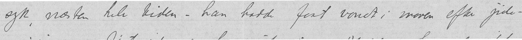syk, næsten hele tiden – han hadde faat vondt i maven efter jule-
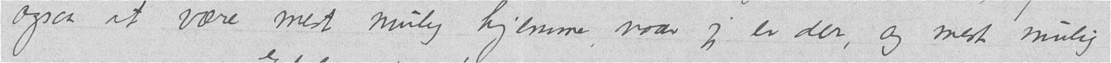ogsaa at være mest mulig hjemme, naar jeg er der, og mest mulig
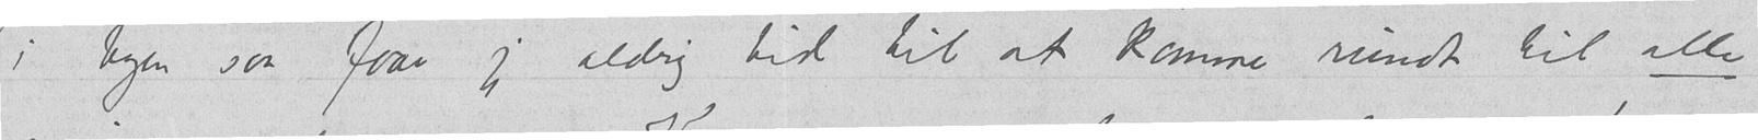i byen saa faar jeg aldrig tid til at komme rundt til alle
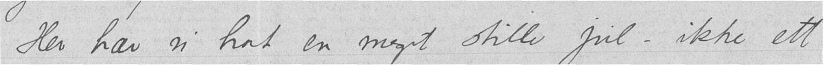Her har vi hat en meget stille jul – ikke ett
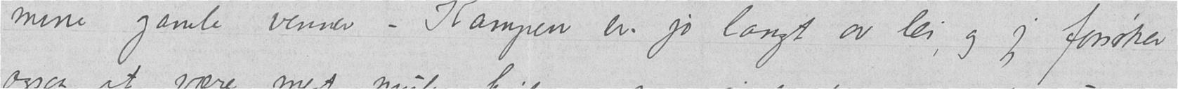mine gamle venner – Kampen er jo langt av lei, og jeg forsøker
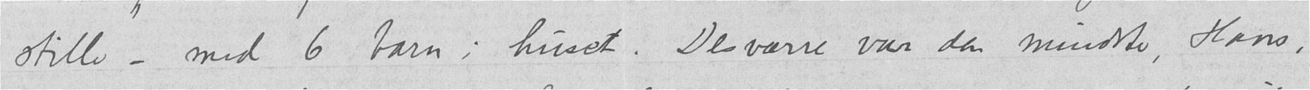stille – med 6 barn i huset. Desværre var den mindste, Hans,
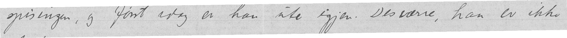spisingen, og først idag er han ute igjen. Desværre, han er ikke
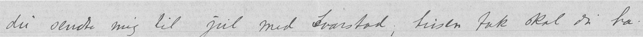du sendte mig til jul med Svarstad; tusen tak skal du ha.
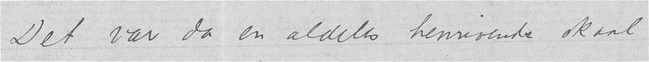Det var da en aldeles henrivende skaal
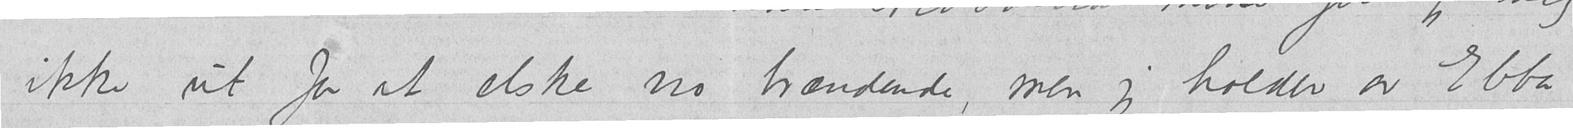ikke ut for at elske no brændende, men jeg holder av Ebba
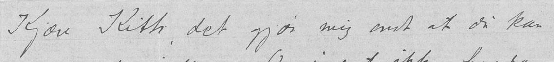Kjære Kitti, det gjør mig ondt at du kan
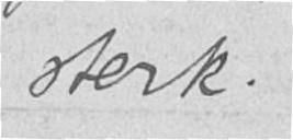sterk.
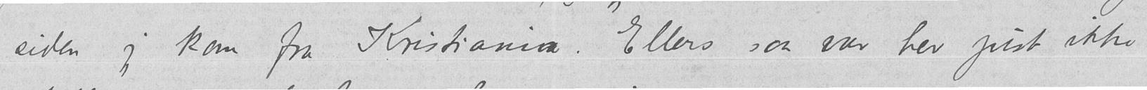siden jeg kom fra Kristiania. Ellers saa var her just ikke
